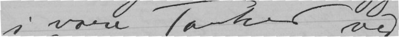i vore Tanker ved
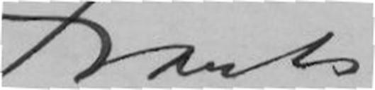Frants
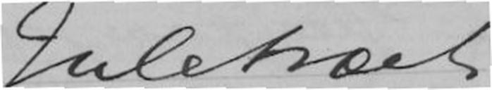Juletræet!
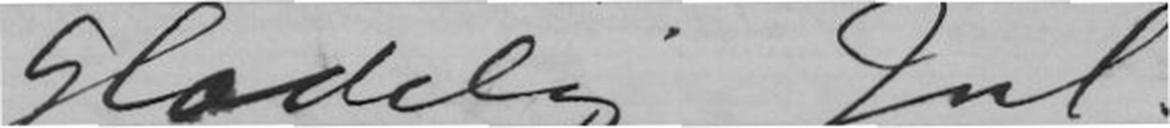Og Glædelig Jul!
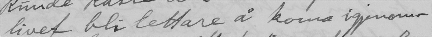livet bli lettare å koma igjenom –
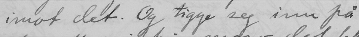imot det. Og tigge seg inn på
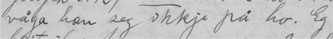våga han seg ikkje på ho. Eg
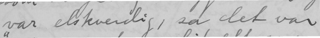var elskverdig, sa det var
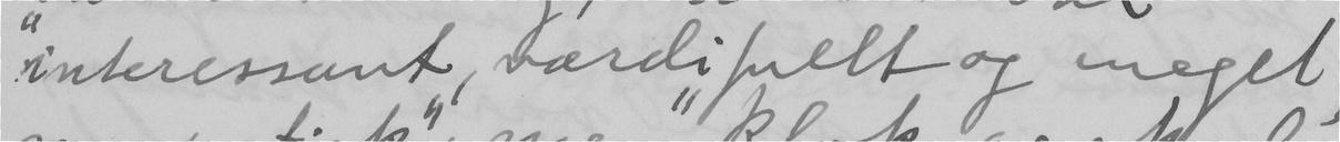"interessant, værdifullt og meget og
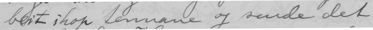beit ihop tennane og sende det
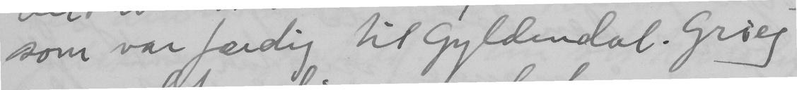som var færdig til Gyldendal. Grieg
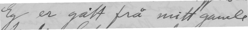Eg er gått frå mitt gamle
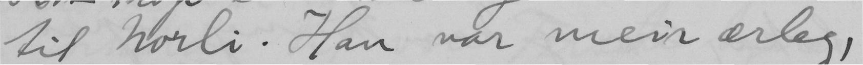til Norli. Han var meir ærleg,
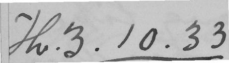Hv. 3.10.33
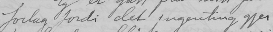forlag fordi det ingenting gjer
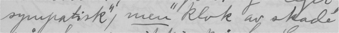sympatisk", men "klok av skade"
